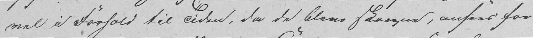vel i Forhold til Tiden, da de bleve skrevne, ansees for
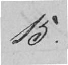15.
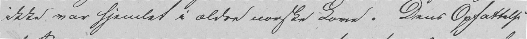ikke var hjemlet i ældre norske Love. Dens Opfattelse
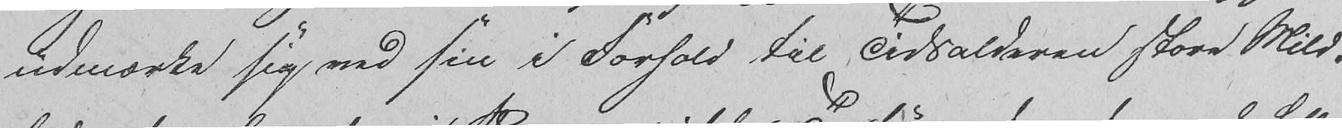udmærke sig ved sin i Forhold til Tidsalderen store Mild-
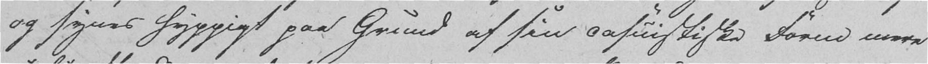og synes hyppigt paa Grund af sin casuistiske Form mere
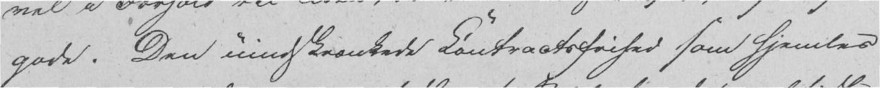gode. Den uindskrænkede Kontractsfrihed som hjemles
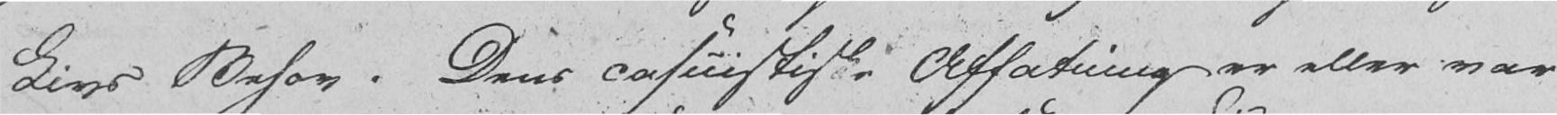Livs Behov. Dens casuistiske Affatning er eller var
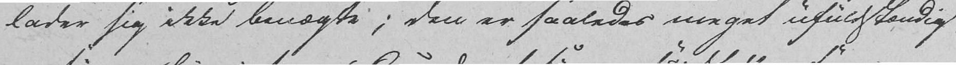lader sig ikke benægte; den er saaledes meget ufuldstændig
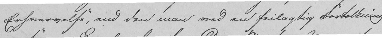Erhvervelse, end den man ved en feilagtig Fortolkning
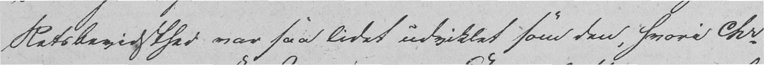Retsbevidsthed var saa lidet udviklet som den, hvori Chr
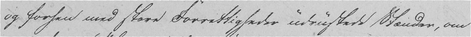og forhen med store Forrettigheder udrustede Stænder, om
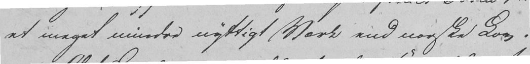et meget mindre nyttigt Værk end norske Lov.
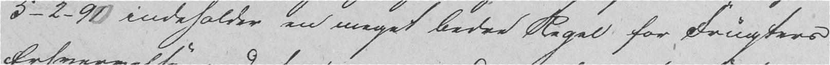5-2-91 indeholder en meget bedre Regel for Frugters
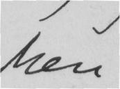Men
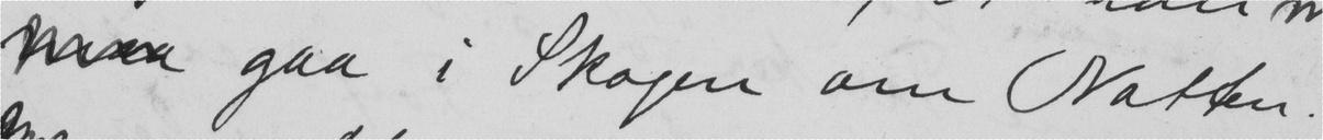gaa i Skogen om Natten.
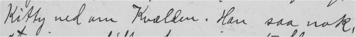Kitty ned om Kvælden. Han saa nok,
